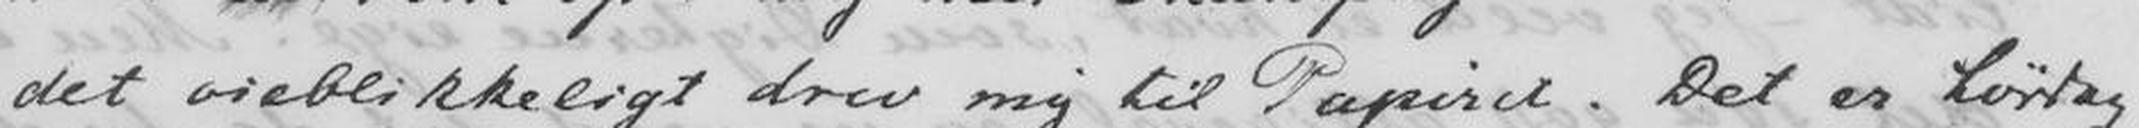det øieblikkeligt drev mig til Papiret. Det er Lørdag
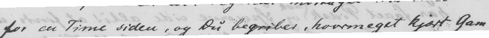for en Time siden, og Du begriber, hvormeget kjært Gam-
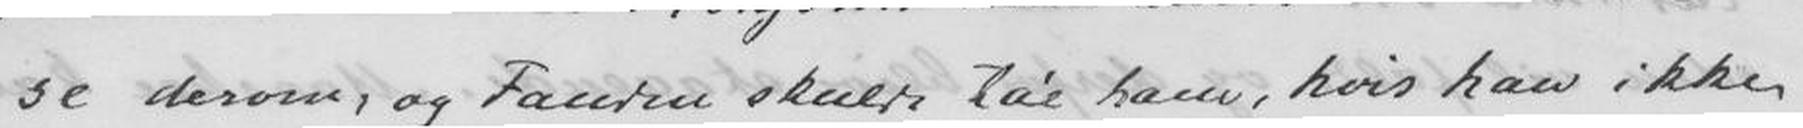se derom, og Fanden skulde ta'e ham, hvis han ikke
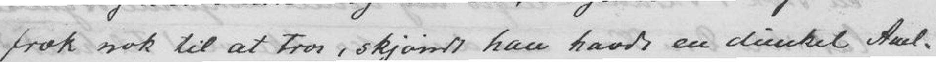fræk nok til at Tro, skjøndt han havde en dunkel Anel-
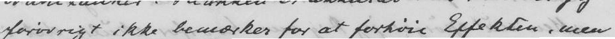forøvrigt ikke bemærker for at forhøre Effekten, men
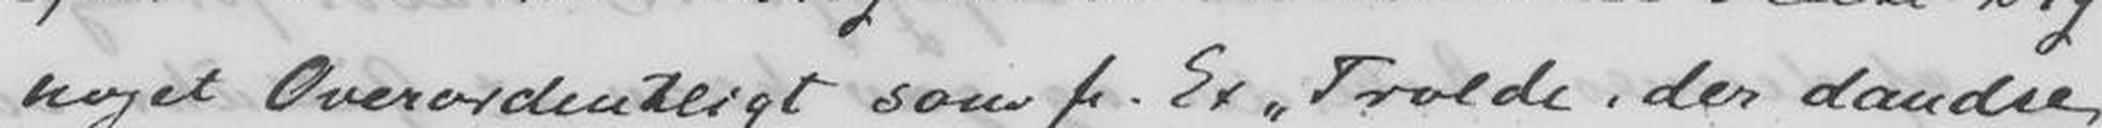noget Overordentligt som f. Ex Trolde, der dandse
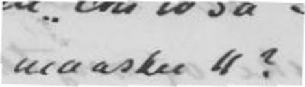maaskee 11?
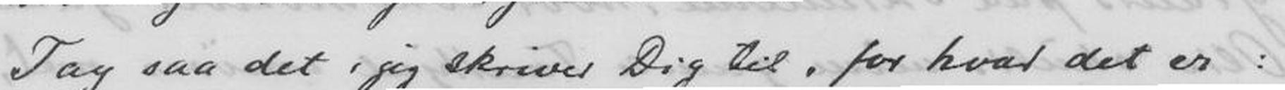Tag saa det, jeg skriver Dig til, for hvad det er:
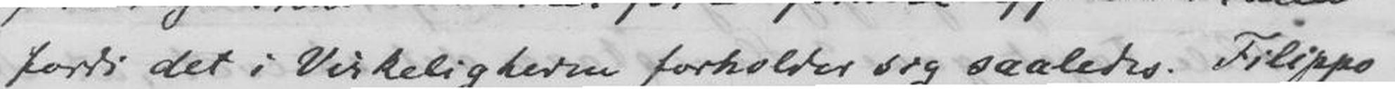fordi det i Virkligheden forholder sig saaledes. Filippo
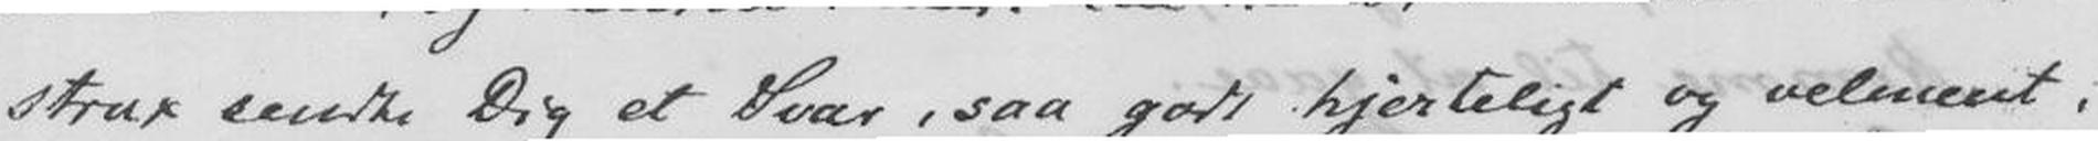strax sendte Dig et Svar, saa godt hjerteligt og velment
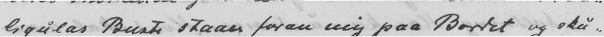ligulas Buste staaer foran mig paa Bordet og sku-
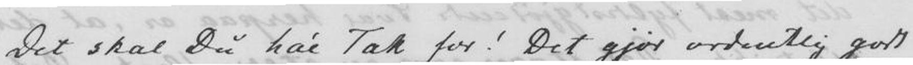Det skal Du ha'e Tak for! Det gjør ordentlig godt
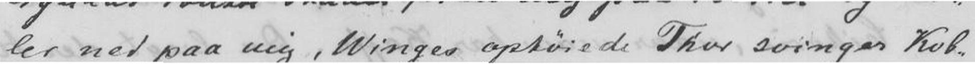ler ned paa mig, Winges optøiede Thor svinger Kob-
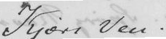Kjære Ven!
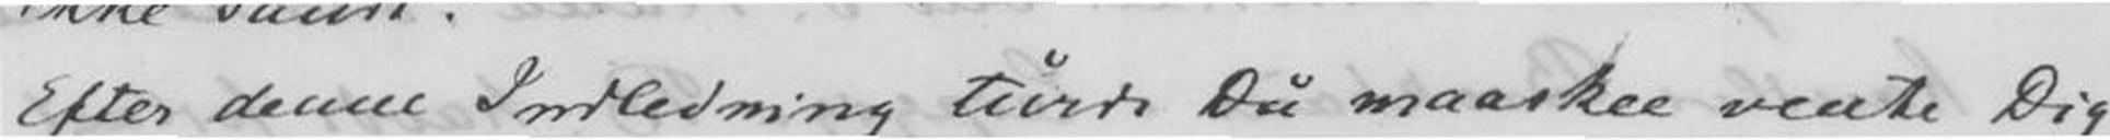Efter denne Indledning turde Du maaskee vente Dig
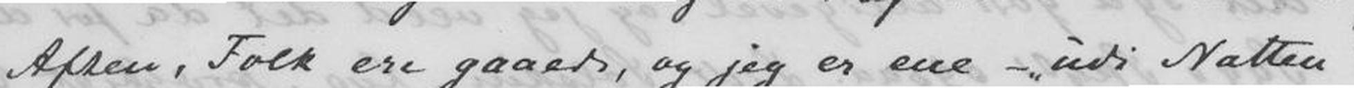Aften, Folk ere gaaede, og jeg er ene - udi Natten
